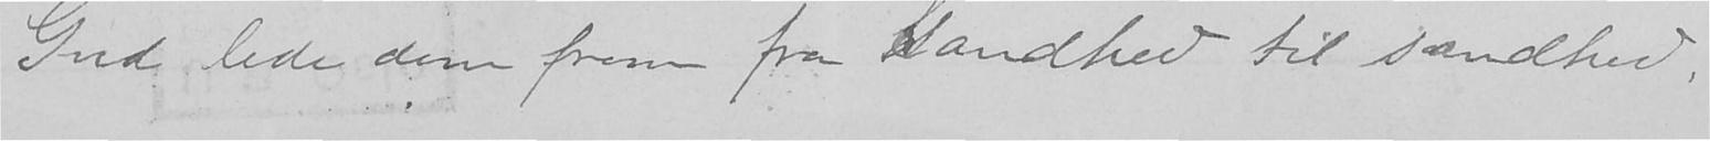Gud lede dem frem fra sandhed til sandhed,
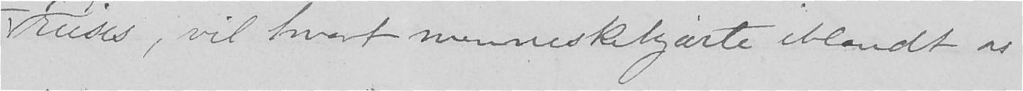reises, vil hvert menneskehjærte iblandt os
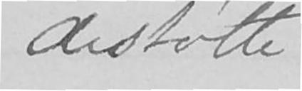destøtte
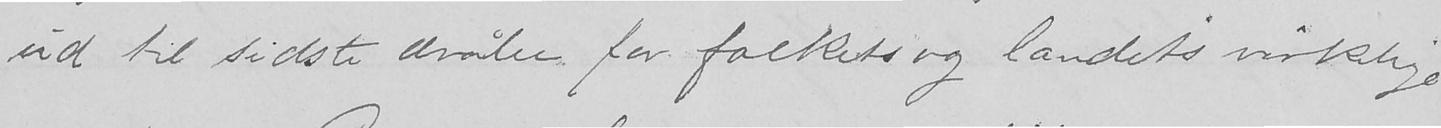ud til sidste dråbe for folkets og landets virkelige
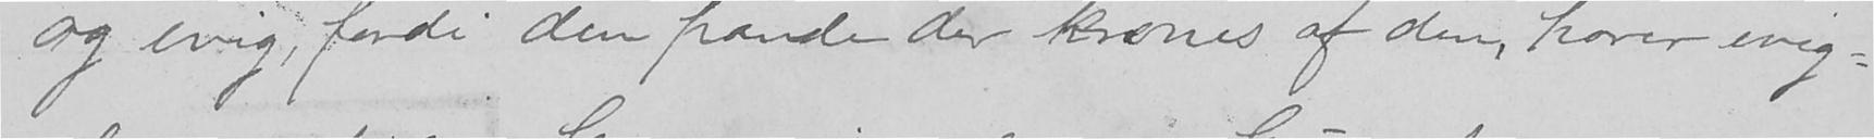og evig, fordi den pande der krones af den, hører evig-
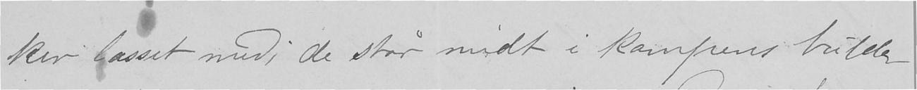ker lasset med; de står midt i kampens bulder
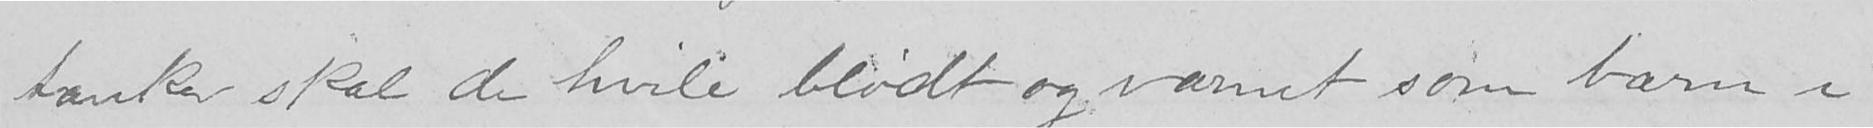tanker skal de hvile blødt og varmt som barn i
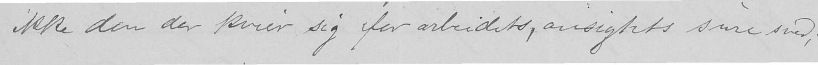ikke den der kvier sig for arbeidets, ansigtets sure sved;
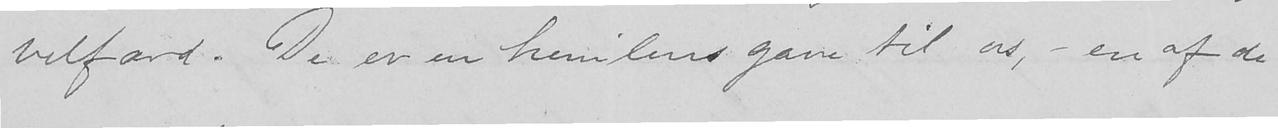velfærd. Du er en himlens gave til os, - en af de
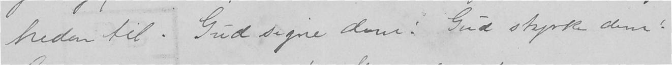heden til. Gud signe dem! Gud styrke dem!
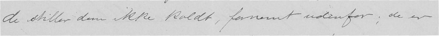de stiller dem ikke koldt, fornemt udenfor; de er
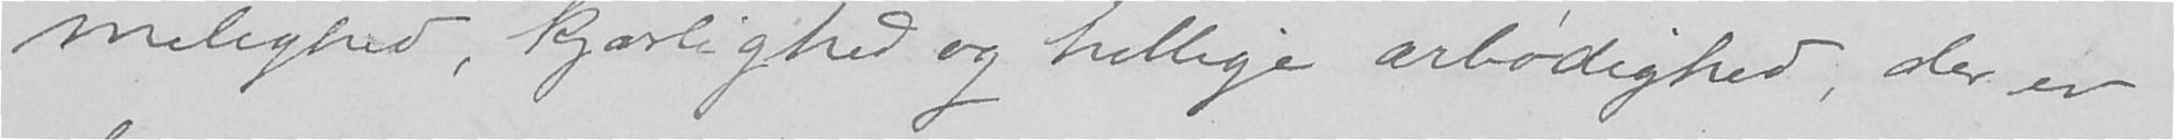melighed, kjærlighed og hellige ærbødighed, der er
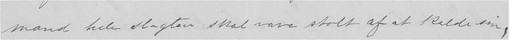mænd hele slægten skal være stolt af at kalde sin,
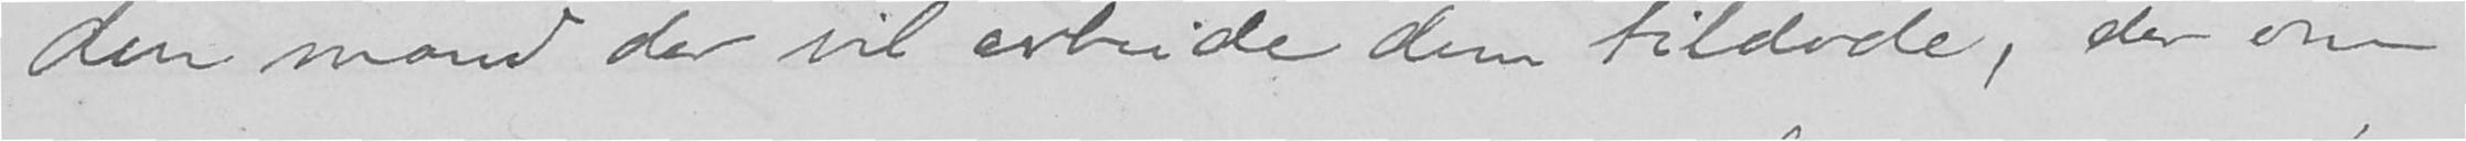den mand der vil arbeide dem tildøde, der om
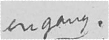engang
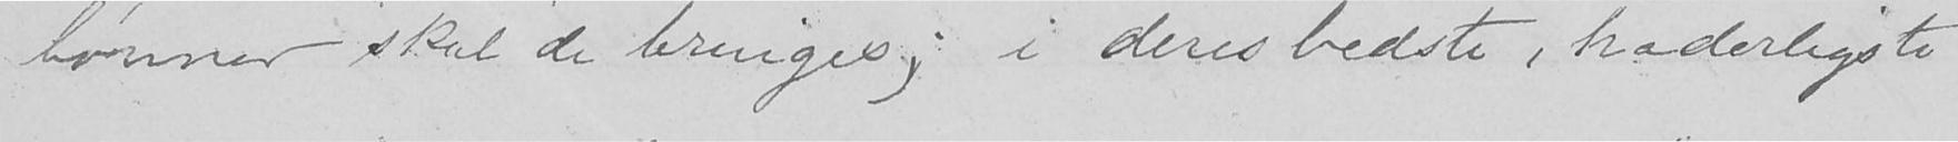bønner skal de bringes; i deres bedste, hæderligste
